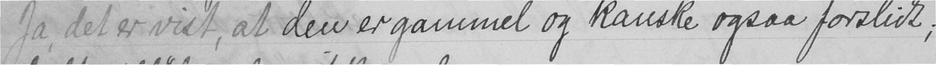Ja det er vist, at den er gammel og kanske ogsaa forslidt;
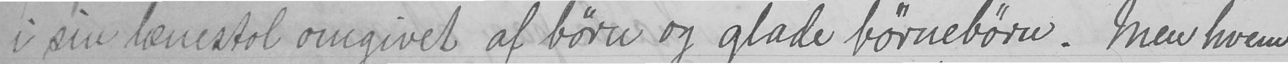i sin lænestol omgivet af børn og glade børnebørn. Men hvem
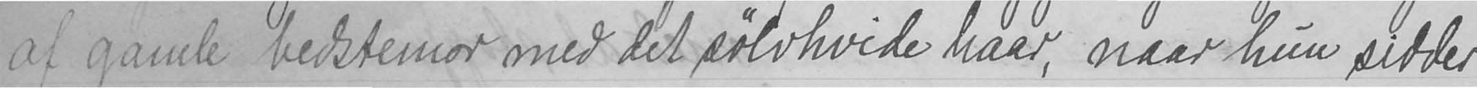af gamle bedstemor med det sølvhvide haar, naar hun sidder
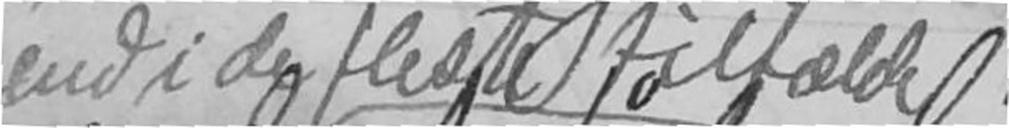end i de fleste tilfælde
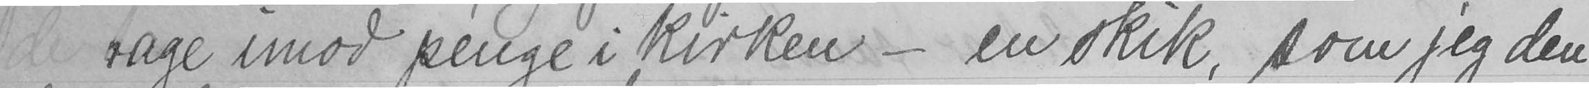de tage imod penge i kirken - en skik, som jeg den
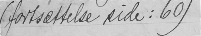(fortsættelse side: 60)
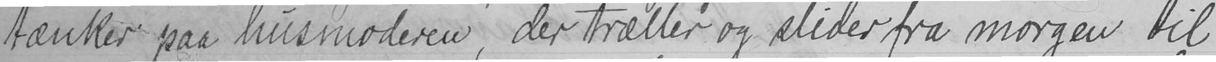tænker paa husmoderen, der træller og slider fra morgen til
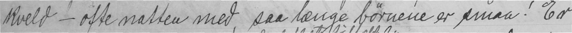kveld - ofte natten med, saa længe børnene er smaa! Er
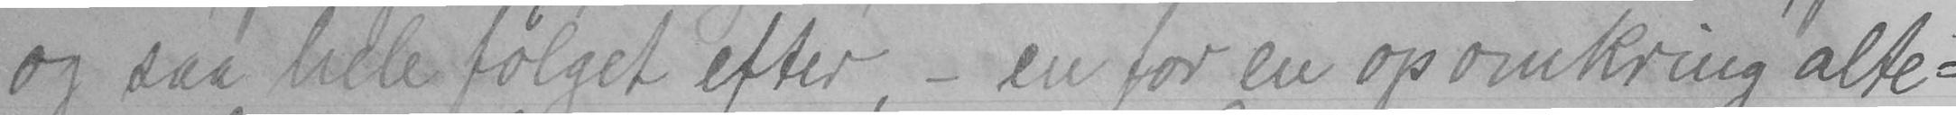og saa hele følget efter, - en for en op omkring alte-
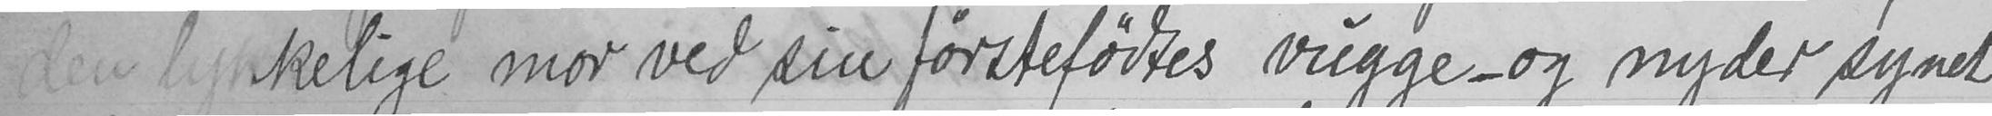den lykkelige mor ved sin førstefødtes vugge- og nyder synet
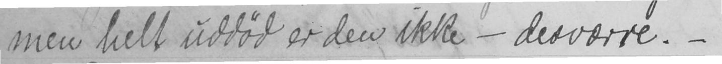men helt uddød er den ikke - desværre. -
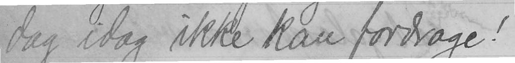dag idag ikke kan fordrage!
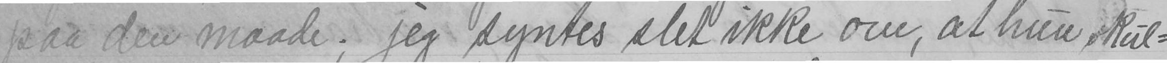paa den maade; jeg syntes slet ikke om, at hun skul-
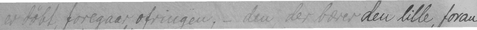er døbt, foregaar ofringen, - den, der bærer den lille, foran
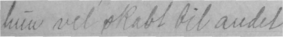hun vel skabt til andet
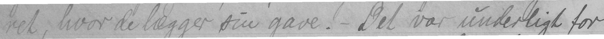ret, hvor de lægger sin gave. - Det var underligt for
